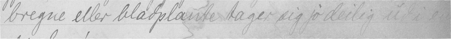bregne eller bladplante tager sig jo deilig ud i en
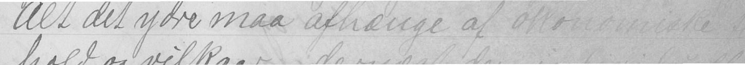alt det ydre maa afhænge af økonomiske
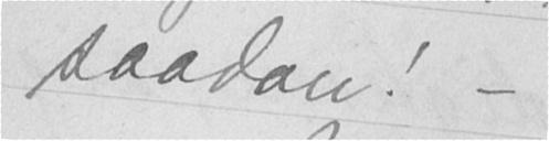saadan! -
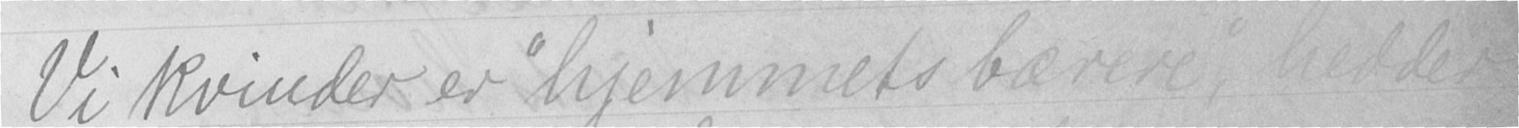Vi kvinder er "hjemmets bærere", hedder
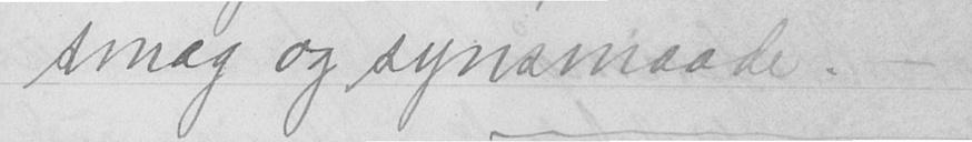smag og synsmaade. -
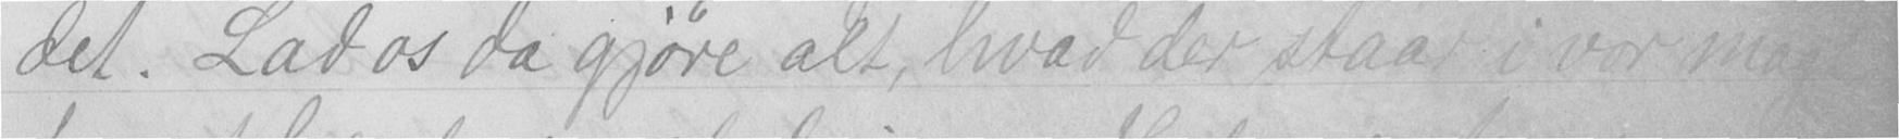det. Lad os da gjøre alt, hvad der staar i vor magt,
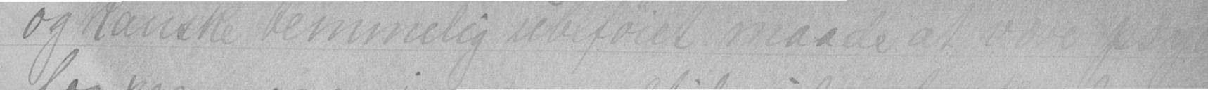og kanske temmelig ubeføiet maade at være
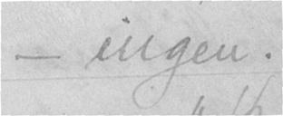- ingen.
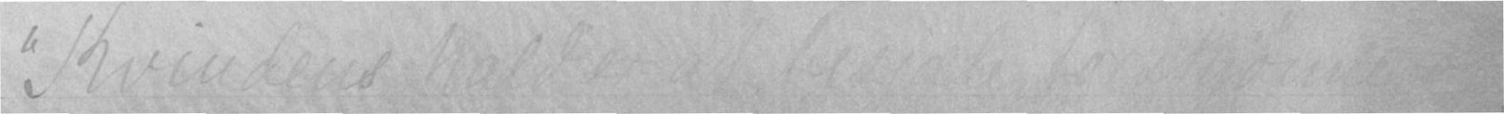"Kvindens  skjønne
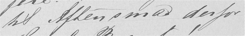til Aftensmad derfor
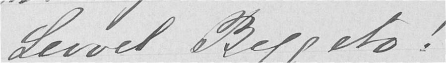Levvel Beggeto!
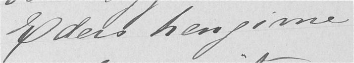Eders hengivne
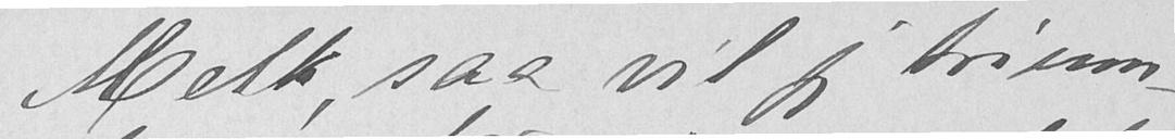Melk, saa vil jeg trium-
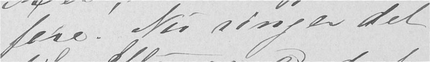fere! Nu ringer det
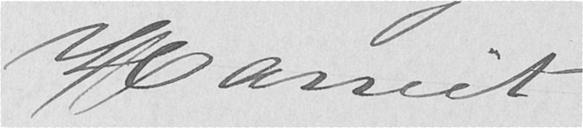Harriet
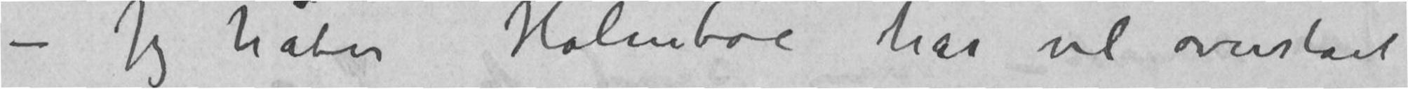– Jeg håber Holmboe har vel overstået
